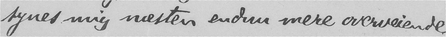synes mig næsten endnu mere overveiende
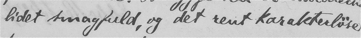lidet smagfuld, og det rent karakterløse
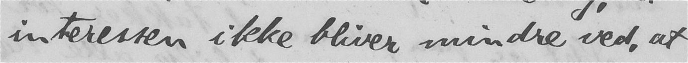interessen ikke bliver mindre ved, at
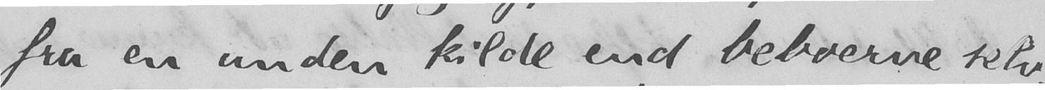fra en anden kilde end beboerne selv.
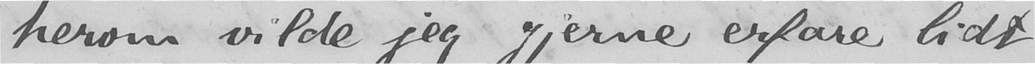herom vilde jeg gjerne erfare lidt
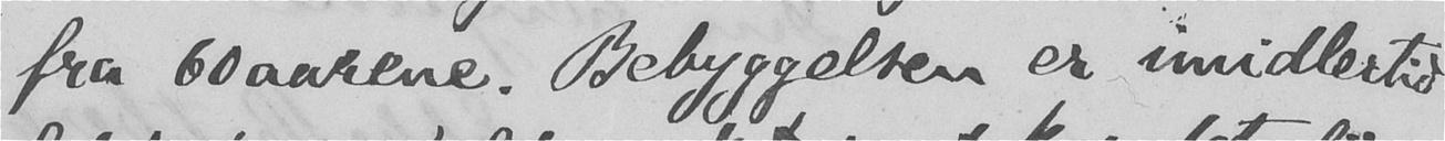fra 60aarene. Bebyggelsen er imidlertid
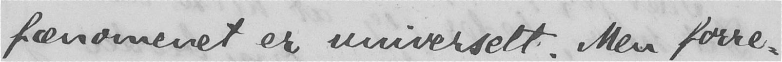fænomenet er universelt. Men forre-
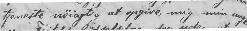tjeneste nøiagtig at opgive mig min unge
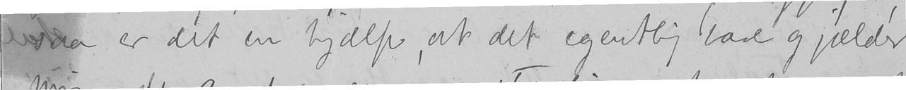saa er det en hjælp, at det egentlig bare gjælder
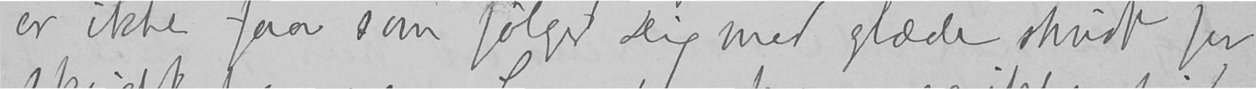er ikke faa som følger Dig med glæde skridt for
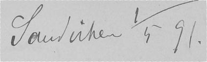Sandviken 1/5 91.
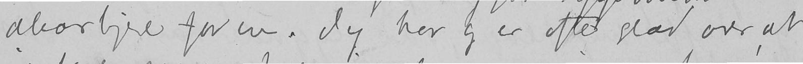alvorligere for en. Jeg har og er ofte glad over, at
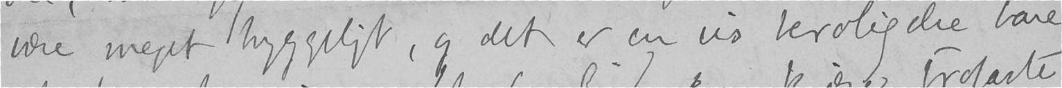være meget hyggeligt, og det er en vis beroligelse bare
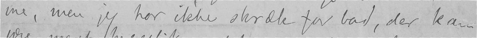me, men jeg har ikke skræk for bad, der kan
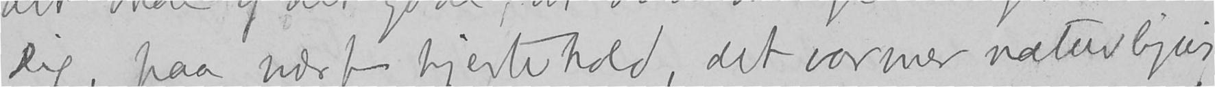Dig, paa nært hjertehold, det varmer naturligvis
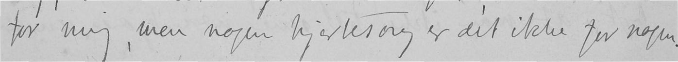for mig, men nogen hjertesorg er det ikke for nogen.
